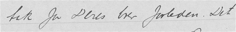tak for Deres brev forleden. Det
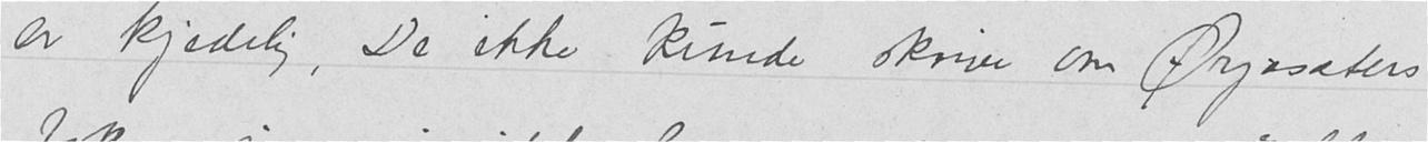er kjedelig, De ikke kunde skrive om Ørjasæters
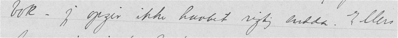bok – jeg opgir ikke haabet rigtig endda.  Ellers
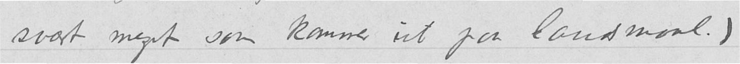svært meget som kommer ut paa landsmaal.)
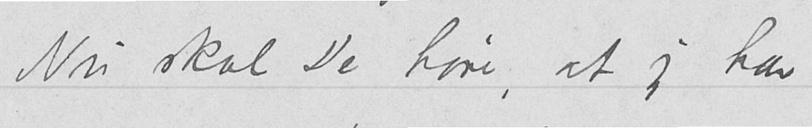Nu skal De høre, at jeg har
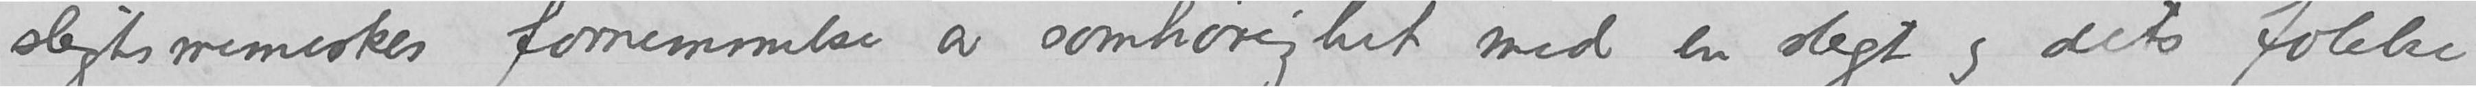slegtsmenneskes fornemmelse av samhørighet med en slegt og dets følelse
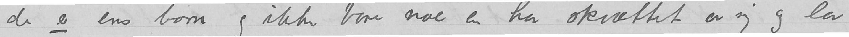de er ens barn og ikke bare noe en har skvættet av sig og lar
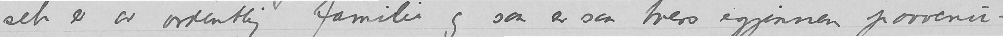selv er av ordentlig familie og saa er saa tvers igjennem parvenu-
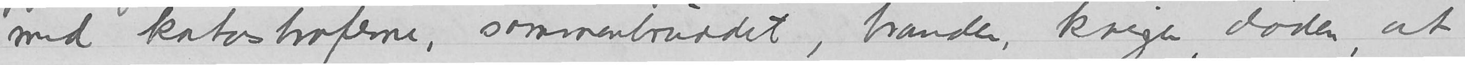med katastroferne, sammenbruddet, brander, kriger, døden, at
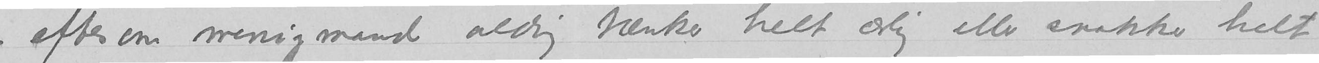- eftersom menigmand aldrig tænker helt ærlig eller snakker helt
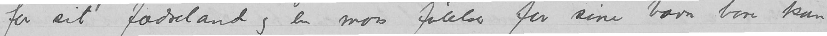for sit fædreland og en mors følelse for sine barn bare kan
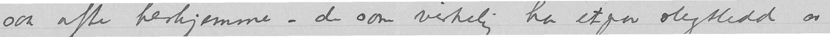saa ofte herhjemme – de som virkelig har etpar slegtledd av
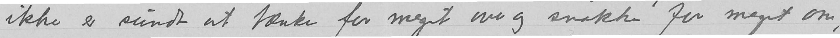ikke er sundt at tænke for meget over og snakke for meget om,
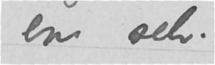en selv.
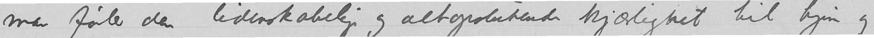man føler den lidenskabelige og altopslukende kjærlighet til hjem og
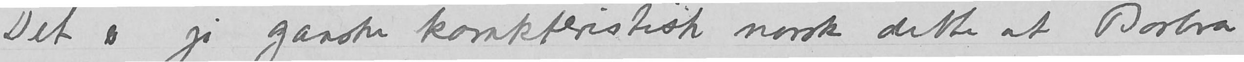Det er jo ganske karakteristisk norsk dette at Barbra
